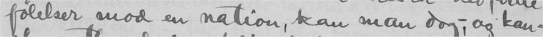følelser mod en nation, kan man dog, og kan-
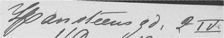Hansteensgd. 2 IV
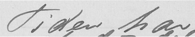Tiden har
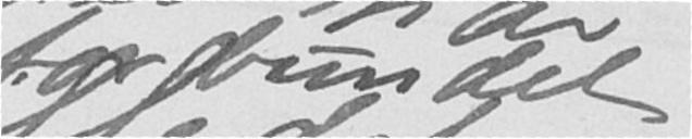forbundet
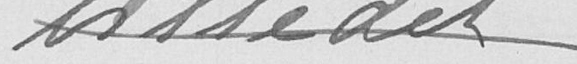billedet
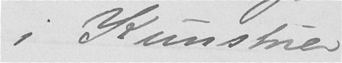i Kunstner
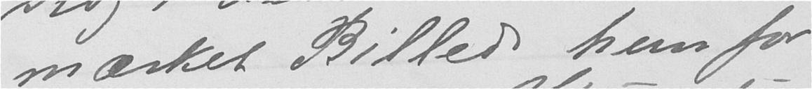mærket Billede, hun for
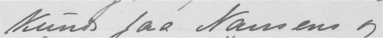kunde faa Nansens og
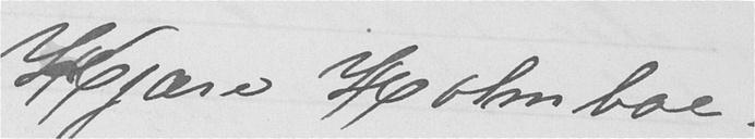Kjære Holmboe.
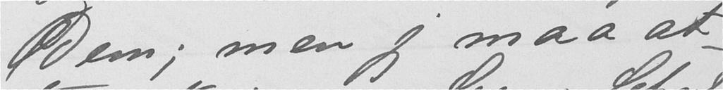Dem; men jeg maa at-
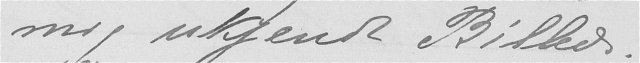mig ukjendt Billede.
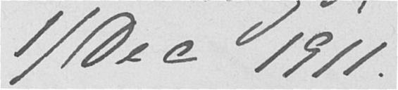11 Dec 1911.
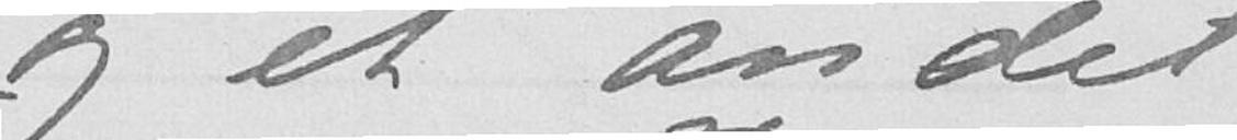og et andet
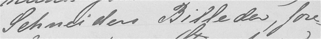Schneiders Billeder, fore-
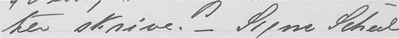ter skrive. - Signe Scheel
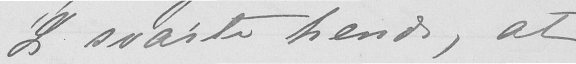Jeg svarte hende, at
